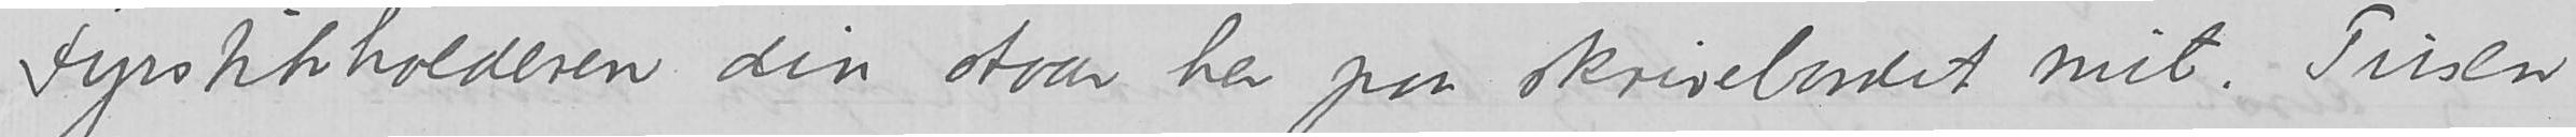Fyrstikholderen din staar her paa skrivebordet mit. Tusen
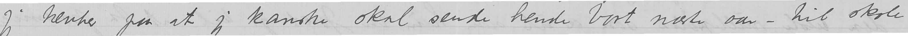jeg tenker paa at jeg kanskje skal sende hende bort næste aar – til skole
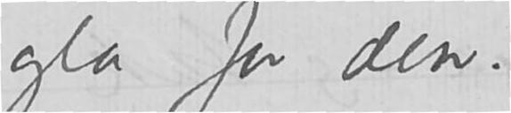gla for den.
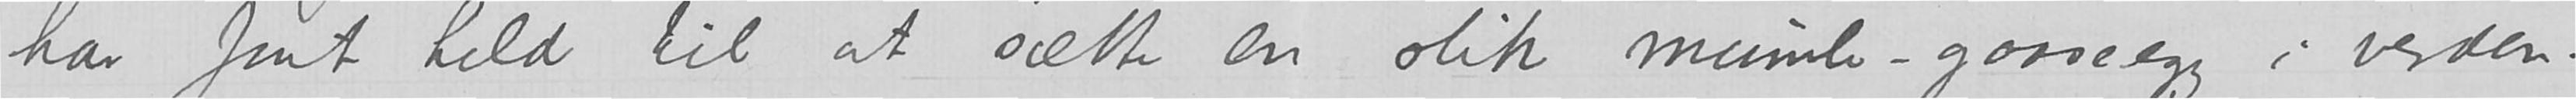har faat held til at sætte en slik mumle-gaaseegg i verden.
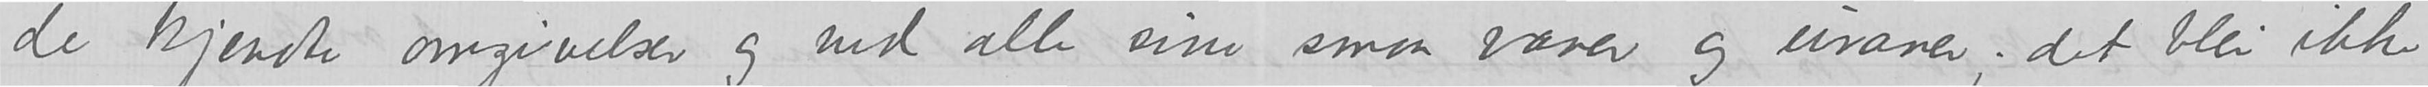mig og ved de kjendte omgivelser og ved alle sine smaa vaner og uvaner; det blir ikke
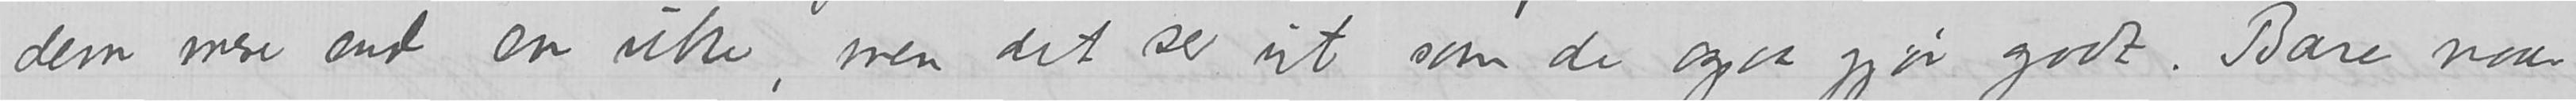dem mere end en uke, men det ser ut som de ogsaa gjør godt. Bare naar
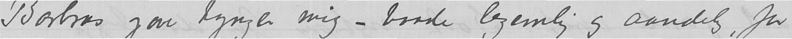-Barbras gave tynger mig – baade legemlig og aandelig, for
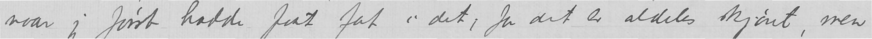naar jeg først hadde faat fat i det, for det er aldeles skjønt, men
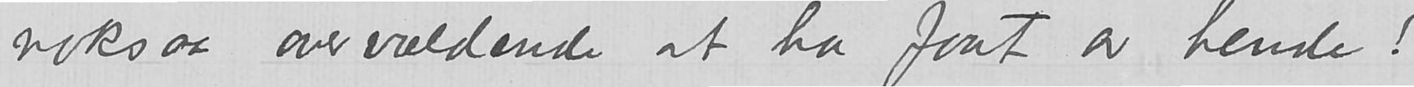noksaa overvældende at ha faat av hende!
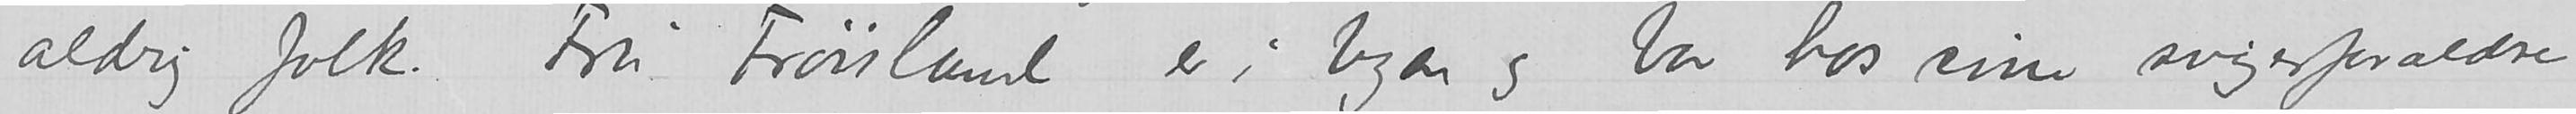aldrig folk. Fru Frøisland er i byen og bor hos sine svigerforeldre
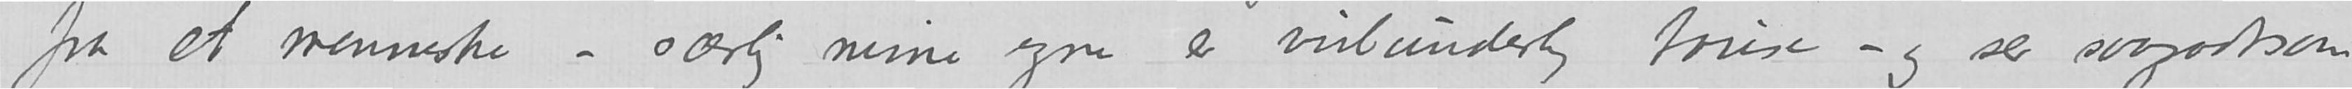fra et menneske – særlig mine egne er vidunderlig tause – og ser saagodtsom
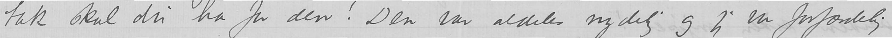tak skal du har for den! Den var aldeles nydelig og jeg er forfærdelig
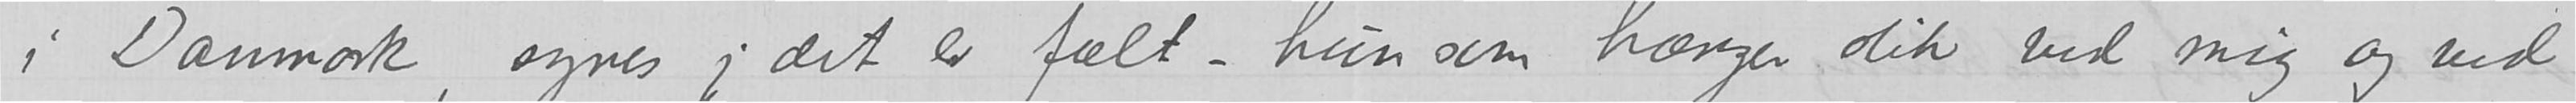i Danmark, synes jeg det er fælt – hun som hænger slik ved
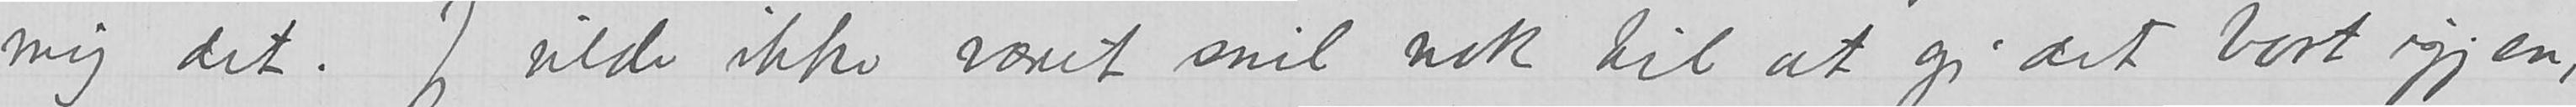mig det. Jeg vilde ikke været snil nok til at gi det bort igjen,
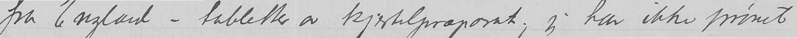fra England – tabletter av kjertelpræparat; jeg har ikke prøvet
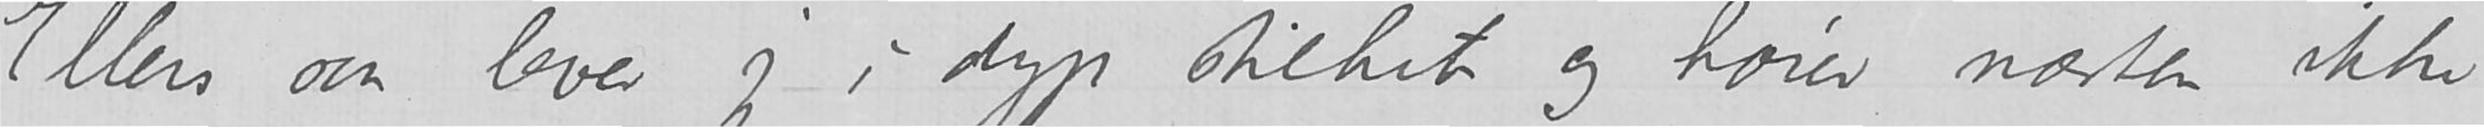Ellers saa lever jeg i dyp stilhet og hører næsten ikke
